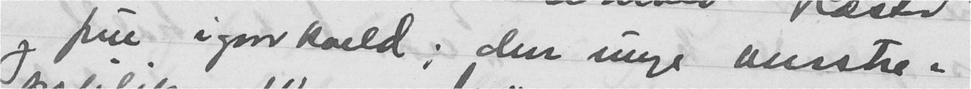og frue igaar kveld; den unge venstre-
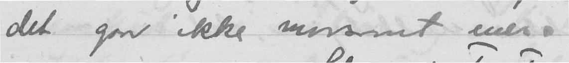det gaar ikke morsomt mer.
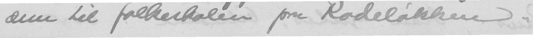dem til folkeskolen paa Rodeløkken.
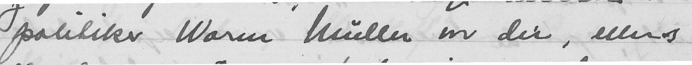politiker Worm Müller var der, ellers
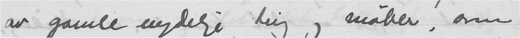av gamle nydelige ting og møbler, som
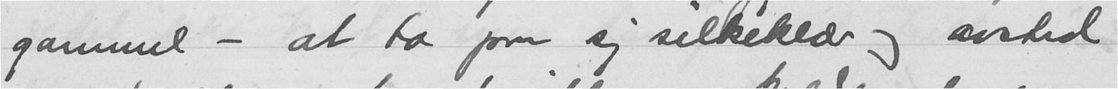gammel - at ta paa sig silkeklær og avsted
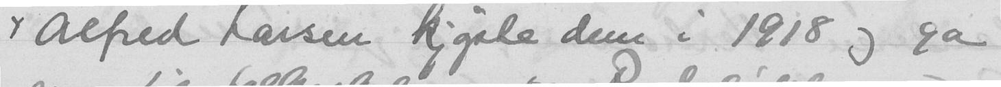*Alfred Larsen kjøpte dem i 1918 og ga
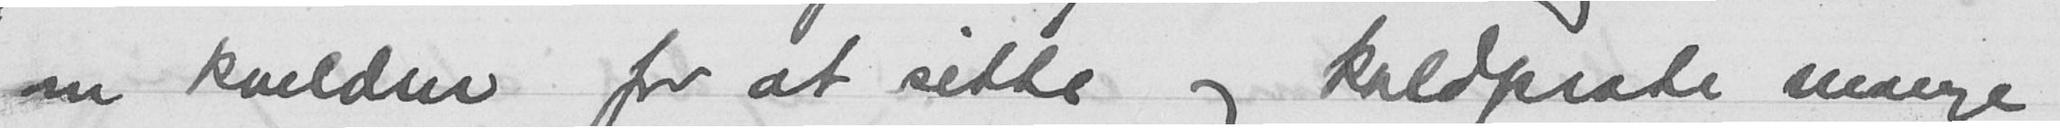om kvelden for at sitte og kaldprate mange
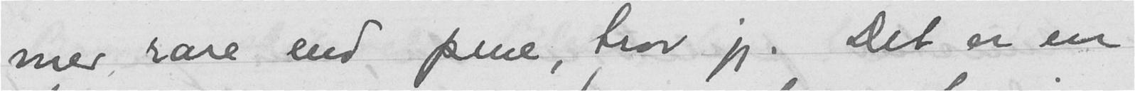mer rare end pene, tror jeg. Det er en
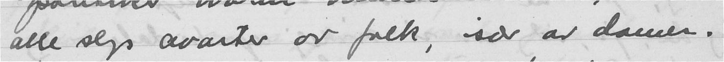alle slags avarter av folk, især av damer.
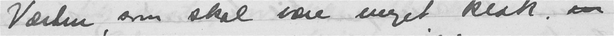Værten, som skal være meget klok, var
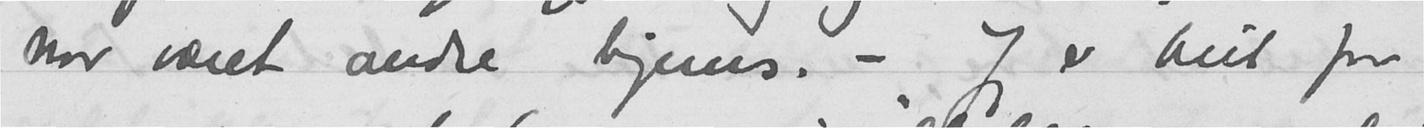har været andre hjems. - J er blit for
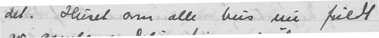det. Huset som alle hus nu fuldt
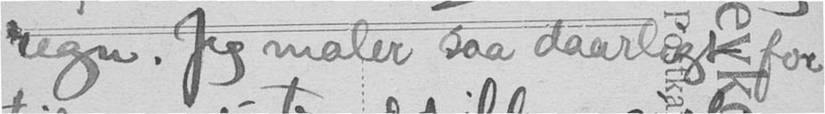regn. Jeg maler saa daarligt for
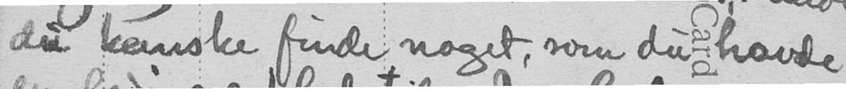du kanske finde noget, som du havde
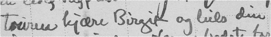touren kjære Birgit og hils din
